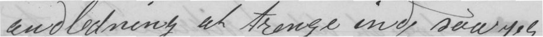andledning at trenge ind, saa jeg
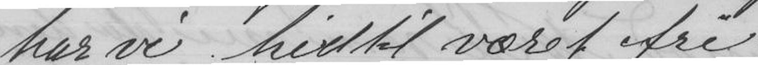har vi hidtil været fri
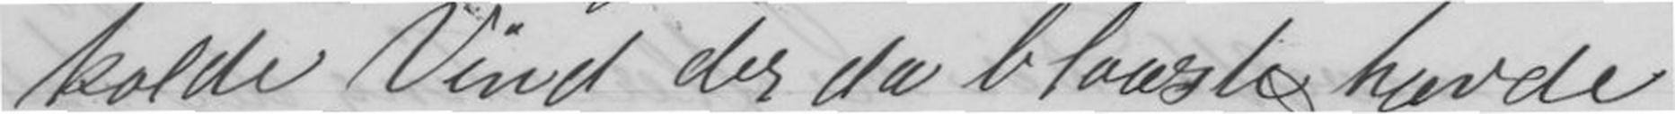kalde Vind der da blaaste havde
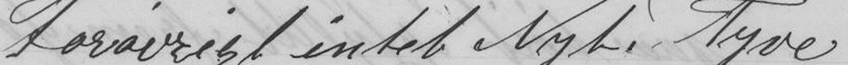Forøvrigt intet Nyt. Tyve
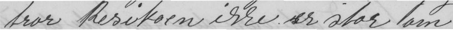tror Risikoen ikke er stor om
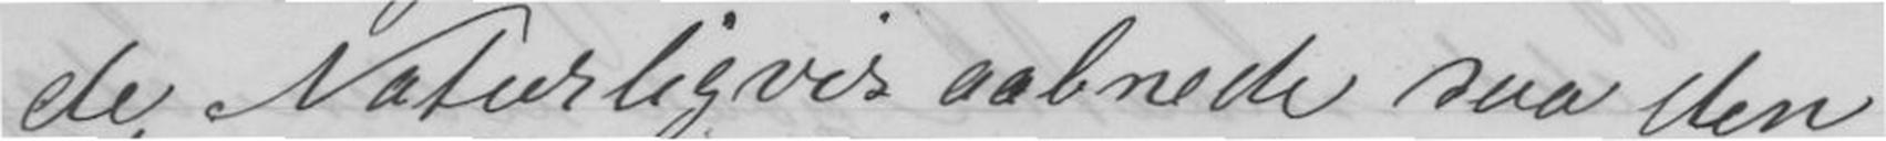de Naturligvis aabnede saa den
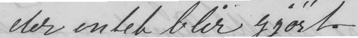der intet blir gjort.
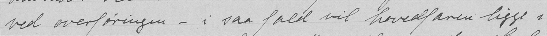ved overføringen - i saa fald vil hovedfaren ligge i
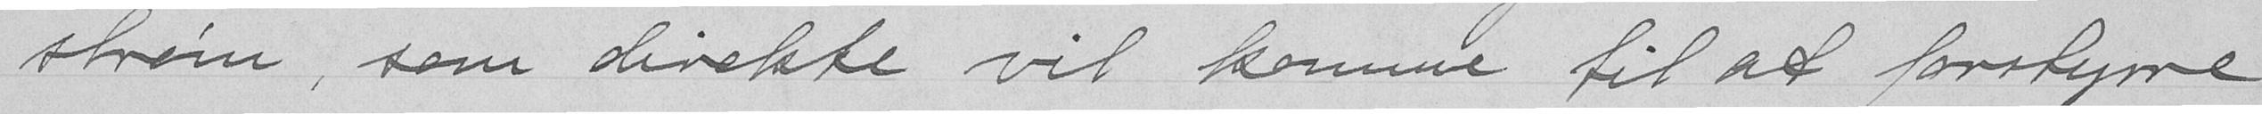strim, som direkte vil komme til at forstyrre
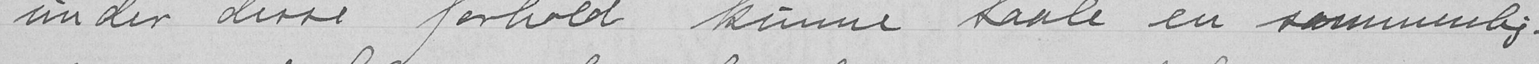under disse forhold kunne taale en sammenlig-
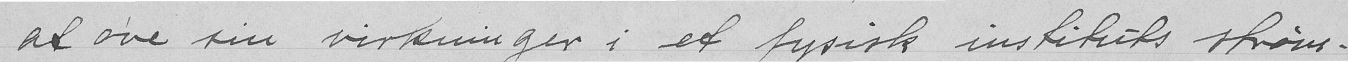at øve sin virkninger i et fysisk instituts strøm-
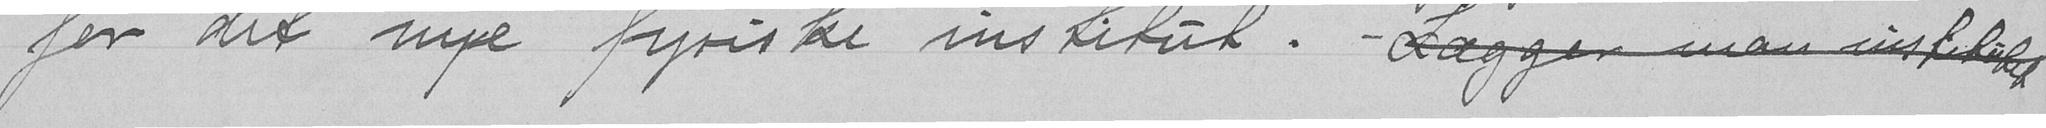for det nye fysiske institut. -Lægger man $
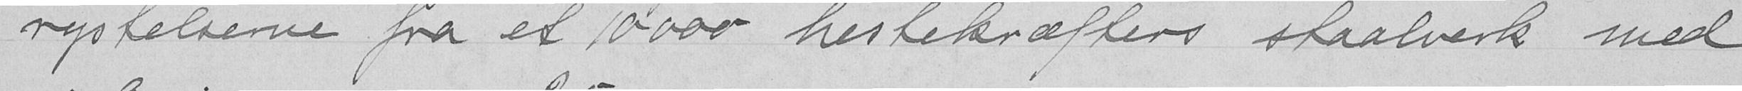rystelserne fra et 10000 hestekræfters staalverk med
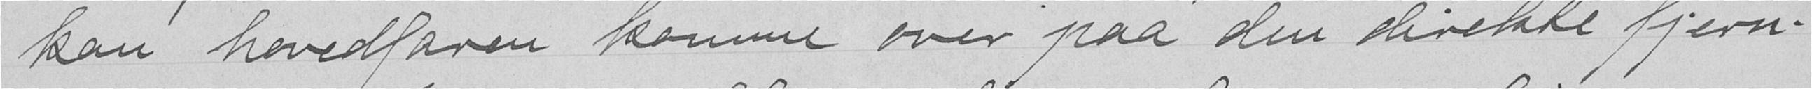kan hovedfaren komme over paa den direkte fjern-
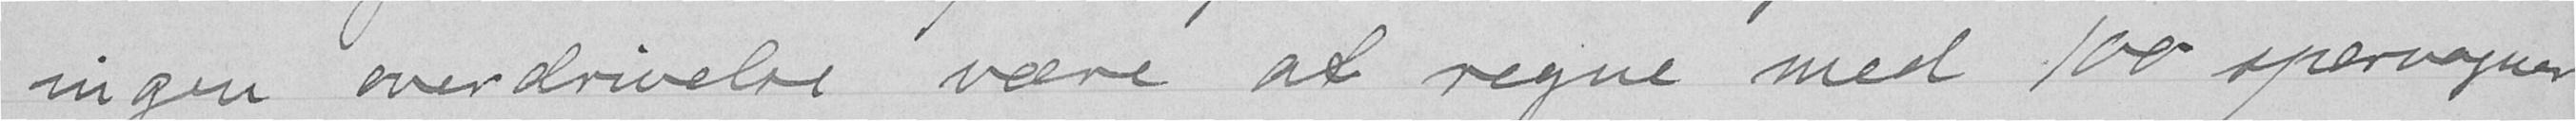ingen overdrivelse være at regne med 100 sporvagner
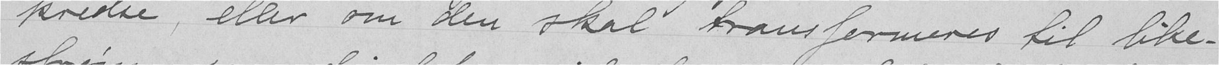kedse, eller om den skal transformeres til like-
